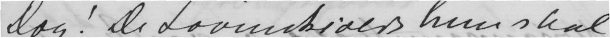Dog! De Løvenskiolds hun skal
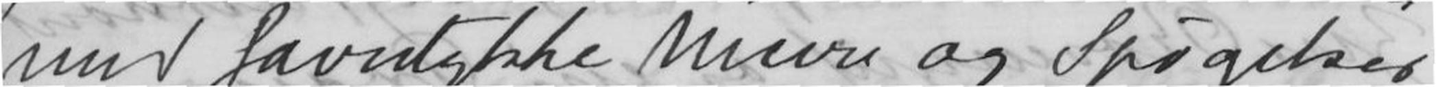med favntykke Mure og og Spøgelser
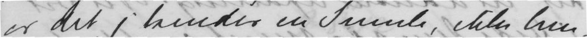er det j kender en Smule, ikke hen-
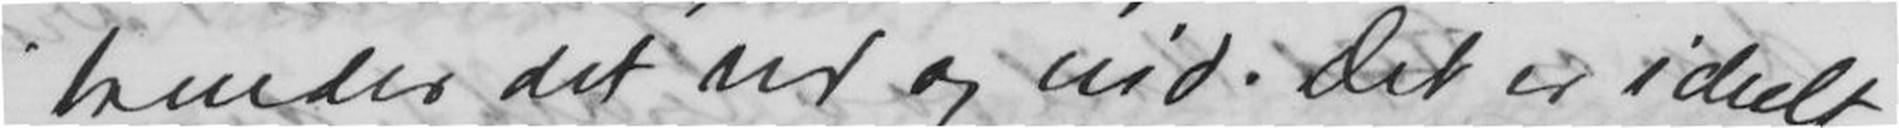j kender det ud og ind. Det er ideelt
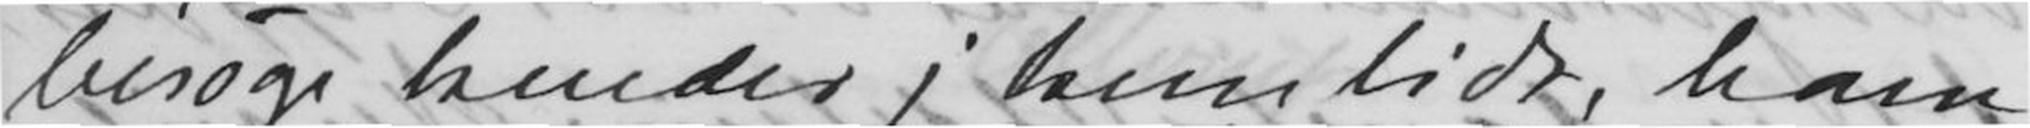besøge kender j kun lidt, ham
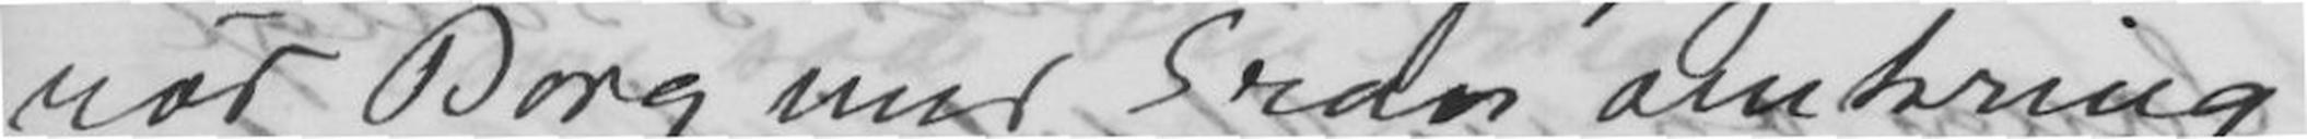rød Borg med Gran omkring
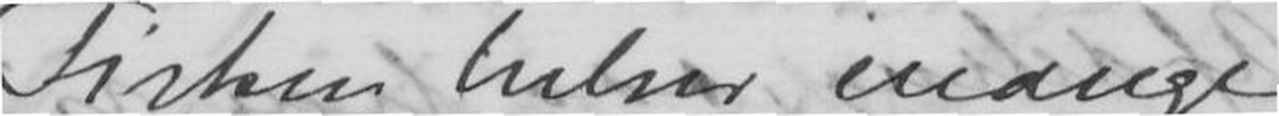Fisken hilser mange
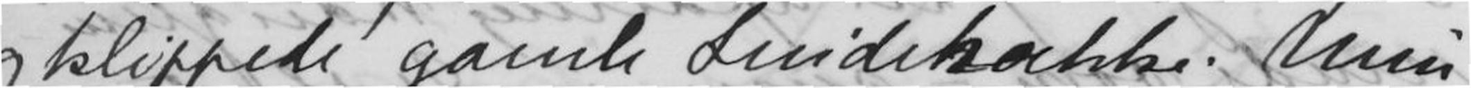og klippede gamle Lindehaekke. Min
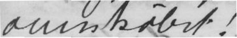ovenikøbet!
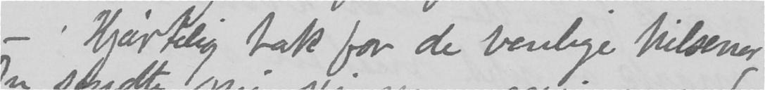Hjærtelig tak for de venlige hilsener
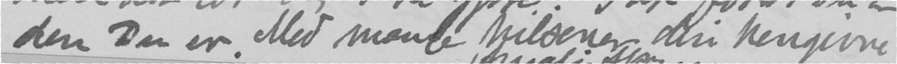den Du er. Med mange hilsener din hengivne
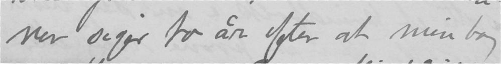ner siger to år efter at min bog
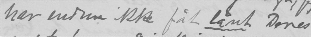har endnu ikke fåt lånt Deres
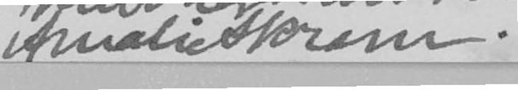Amalie Skram.
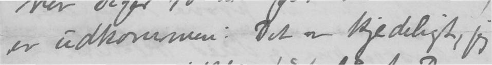er udkommen: Det er kjedeligt, jeg
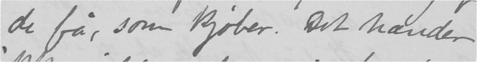de få, som kjøber. Det hænder
